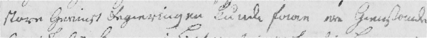store Gevinst Regieringen kunde faae er Genstande
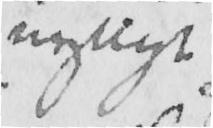nyttigt
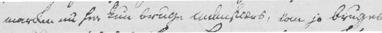marken nu her kun bruges indenskiærs, kan jo bruges
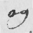og
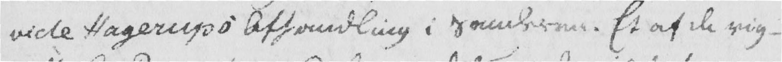vide Hagerups Afhandling i Samleren. Et af de vig-
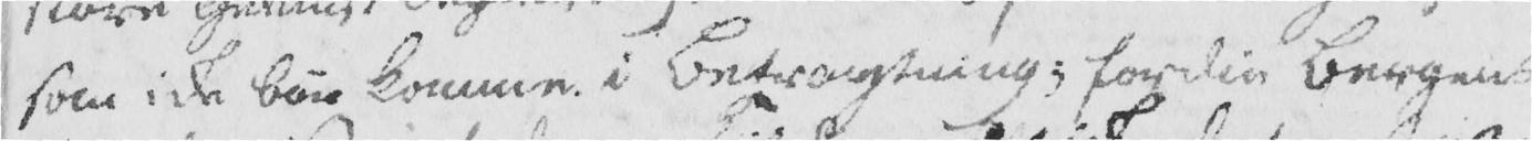som icke bør komme i Betragtning; fordi Bergen
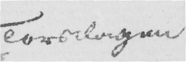Torsdagen
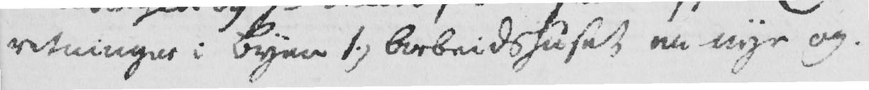retninger i Byen 1) Arbeidshuset en nye og
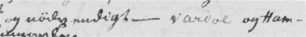og nødvendigt - Vardøe og Ham-
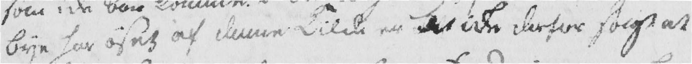Bye har øset af denne Kilde er det icke derfor sagt at
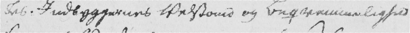kes. Indbyggernes Velstand og Beqvemmelighed
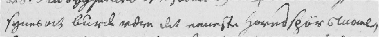synes at burde være det eeneste Hovedspørgsmaal,
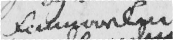Finmarken
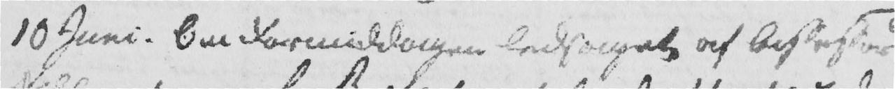10 Juni. Om Formiddagen ledsaget af Assesor
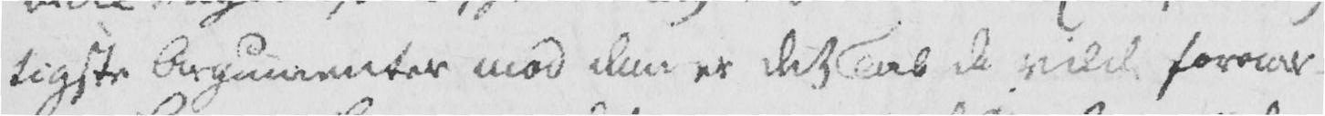tigste Argumenter mod dem er det Tab de vilde foraar-
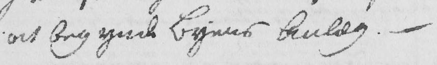at begynde Byens Anlæg. -
